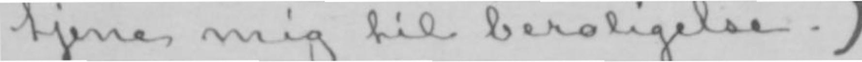tjene mig til beroligelse.
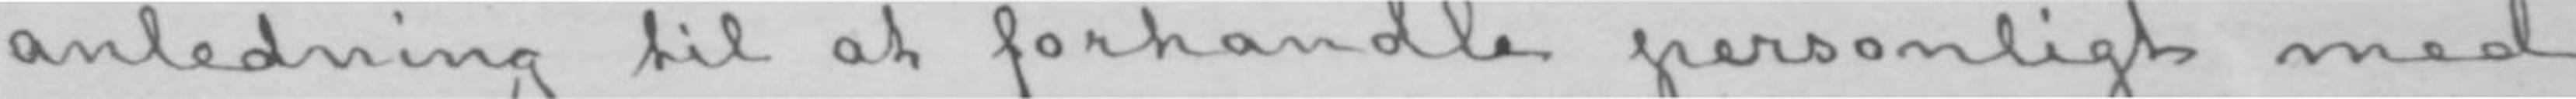anledning til at forhandle personligt med
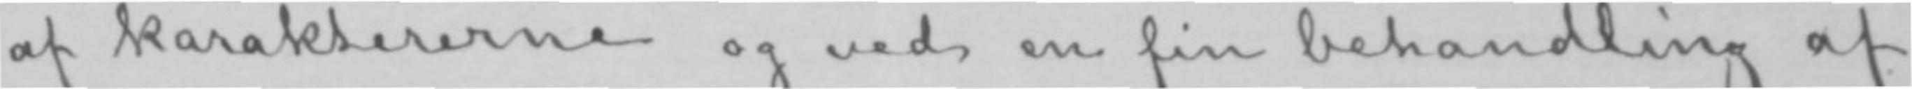af karaktererne og ved en fin behandling af
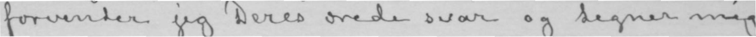forventer jeg Deres ærede svar og tegner mig
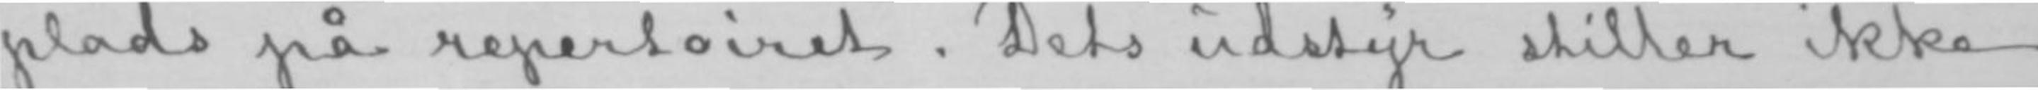plads på repertoiret. Dets udstyr stiller ikke
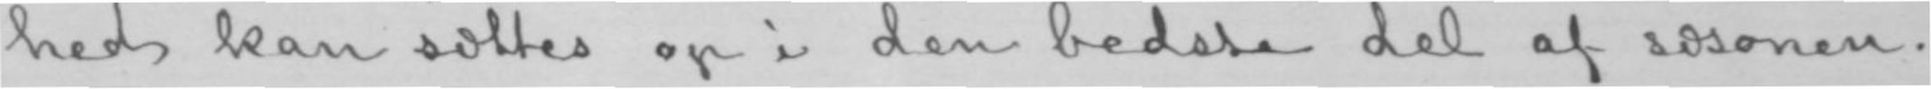hed kan sættes op i den bedste del af sæsonen.
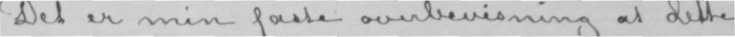Det er min faste overbevisning at dette
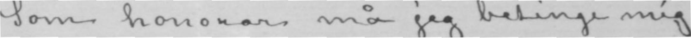Som honorar må jeg betinge mig
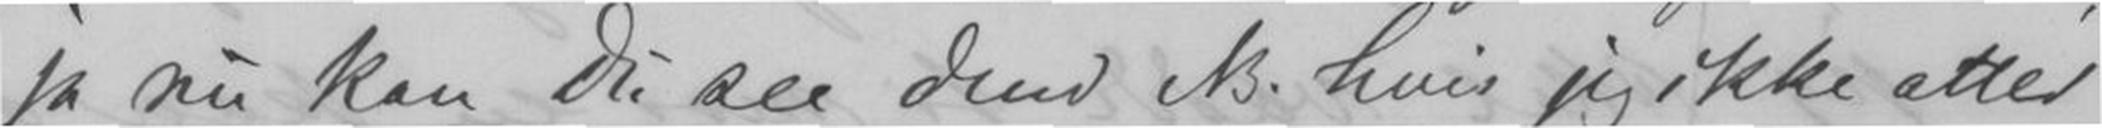ja nu kan Du se dem NB. hvis jeg ikke atter
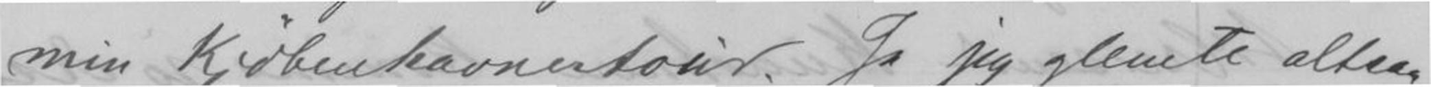min Kjøbenhavnertour. Ja jeg glemte altsaa
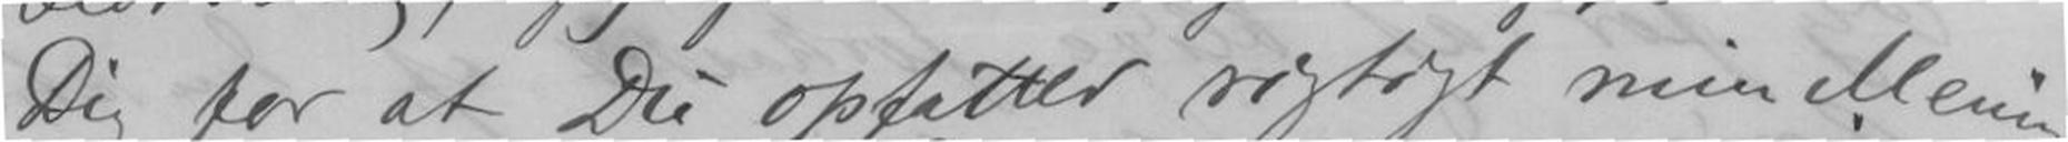Dig for at Du opfatter rigtigt min Mening
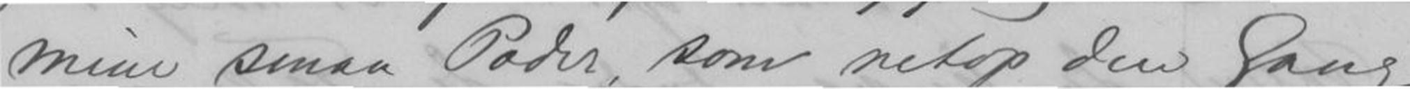mine smaa Poder som netop den Gang
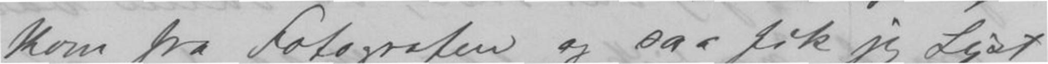kom fra Fotografen og saa fik jeg Lyst
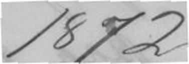1872
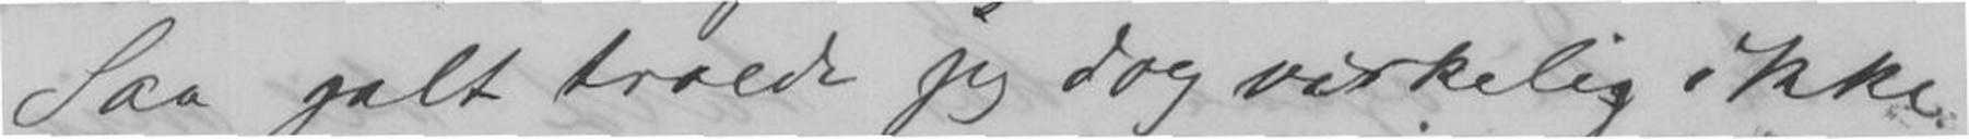Saa galt troede jeg dog virkelig ikke
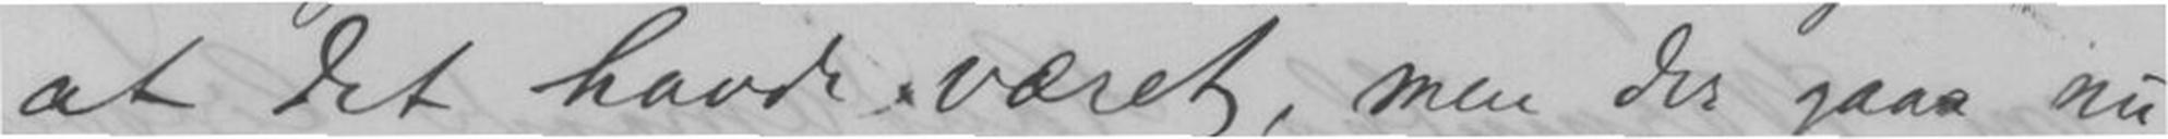at det havde været, men det gaar nu
